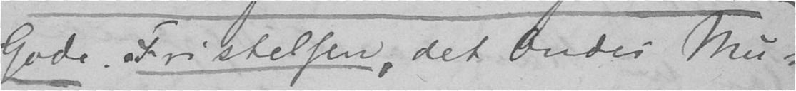Gode. Fristelsen, det Ondes Mu-
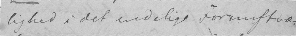lighed i det endelige Fornuftvæ-
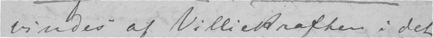vindes af Villieskraften i det
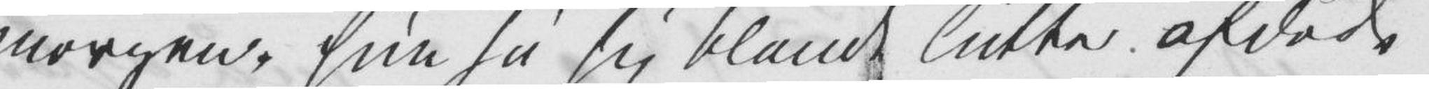morgen. Hun så sig blandt lutter afdøde
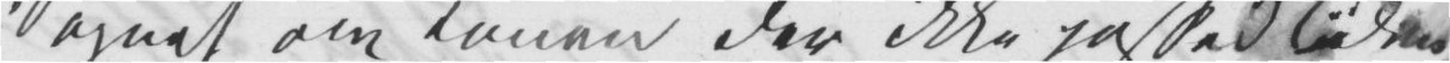Sagnet om Konen der ikke passed Tiden
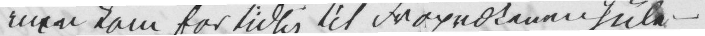men kom for tidlig til Froprækenen Jule¬
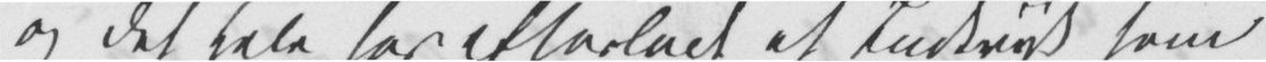og det hele har efterladt et Indtryk som
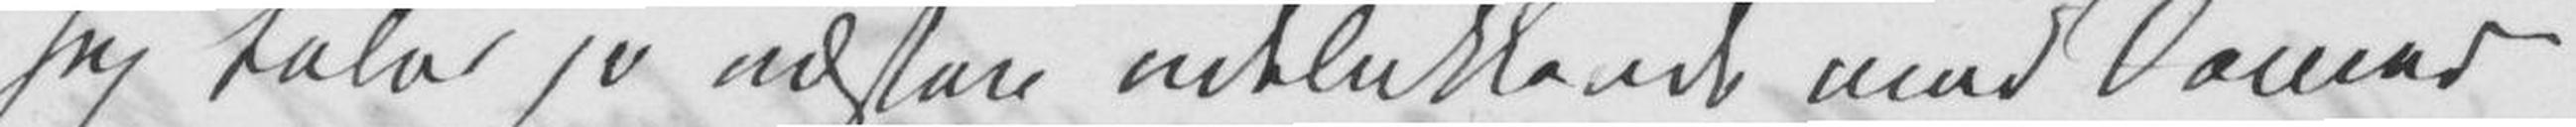Jeg taler jo næsten udelukkende med Damer
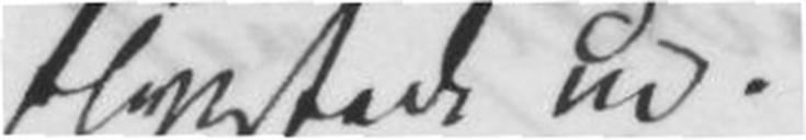flygtede ud.
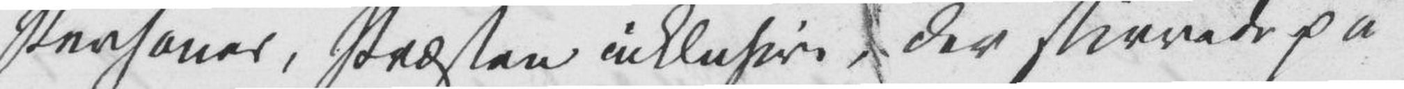Personer, Præsten inklusiv, der stirrede på
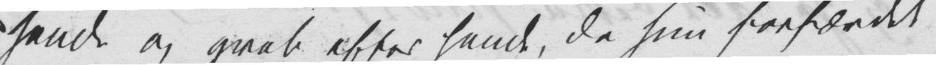hende og greb efter hende, da hun forfærdet
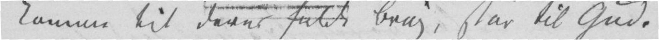komme til deres fulde Brug, står til Gud.
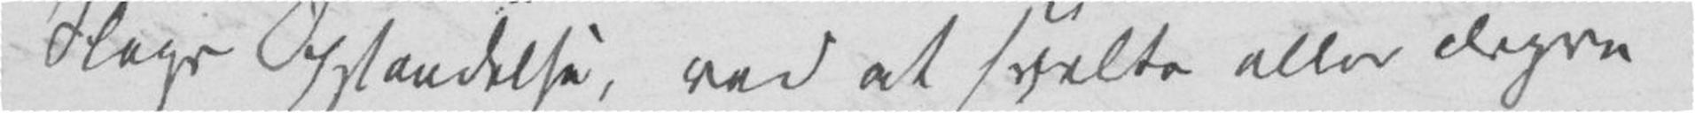Slags Opstandelse, ved at svelte eller ærgre
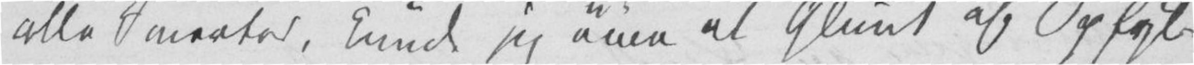alle Smerter, kunde jeg øine et Glimt af Opfyl¬
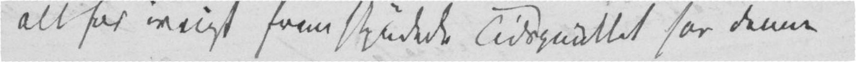alt for ivrigt fremskyndede Tidspunktet for denne
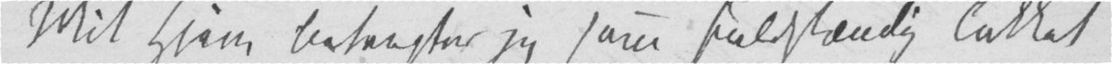Mit Hjem betragter jeg som fuldstændig lukket
